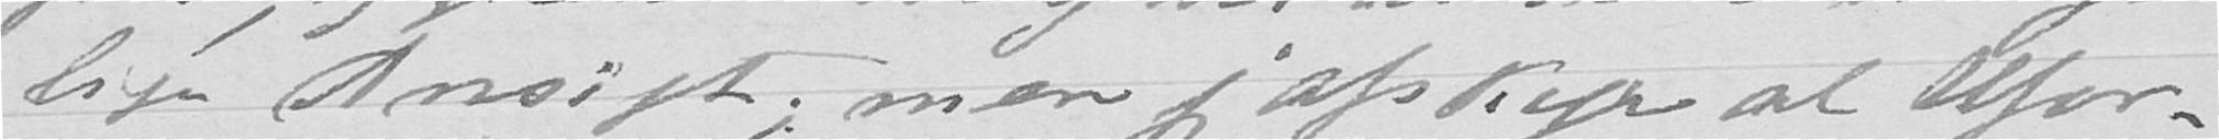lige Ansigt; men jeg afskyr al Ufor-
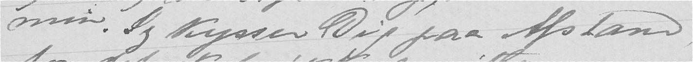min. Jeg kysser Dig paa Afstand
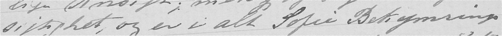sigtighet, og er i alt Sofie Bekymrings
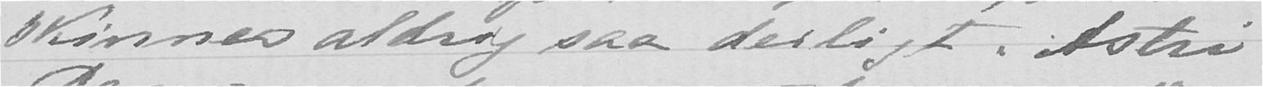skinner aldrig saa deiligt. Astri
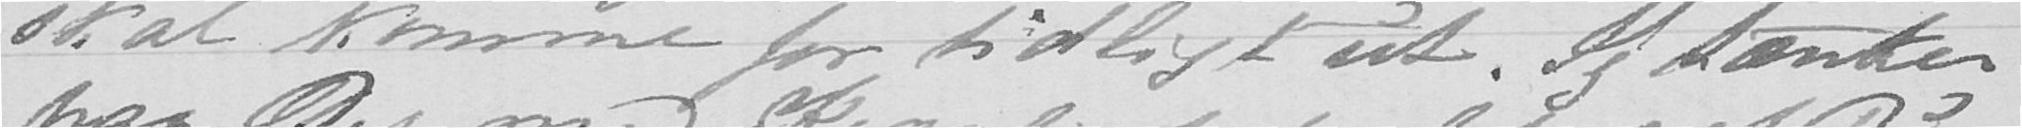skal komme for tidligt ut. Jeg tænker
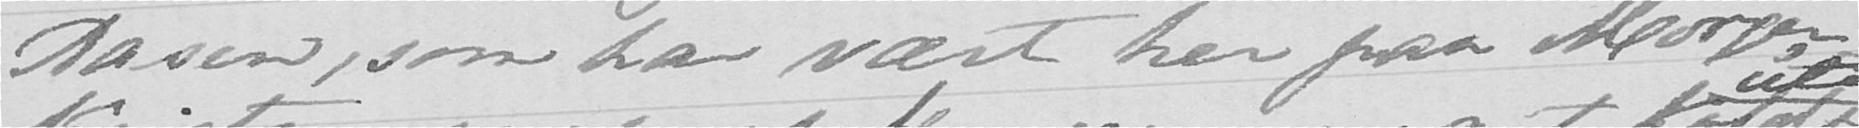Aasen, som har vært her paa Morgen
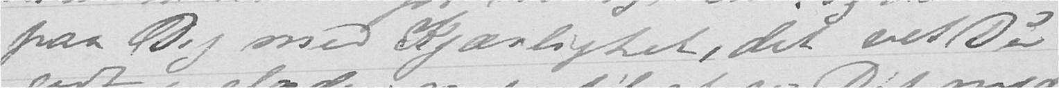paa Dig med Kjærlighet, det vet Du
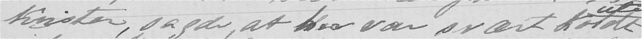Kvisten, sagde, at her var svært koldt
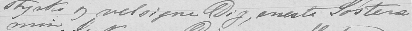styrke og velsigne Dig, eneste Søstera
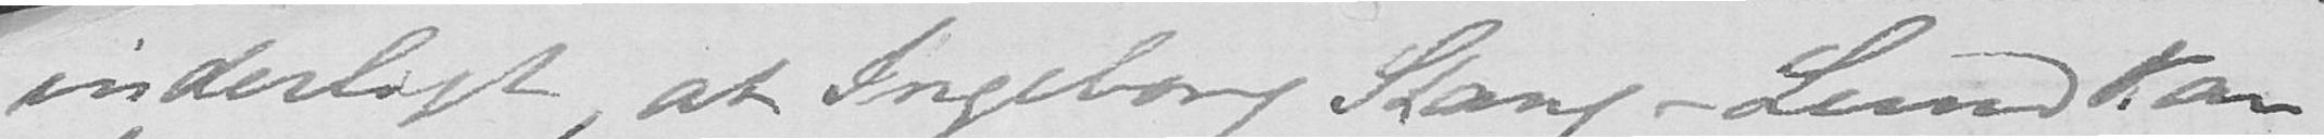inderligt at Ingeborg Stang-Lund kan
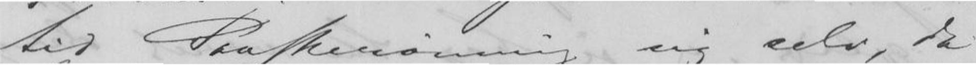tid Paaskerømming sig selv, da
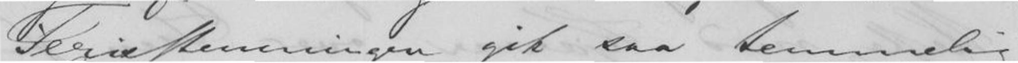Feriestemningen gik saa temmelig
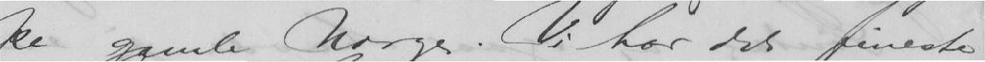pe gamle Norge. Vi har det fineste
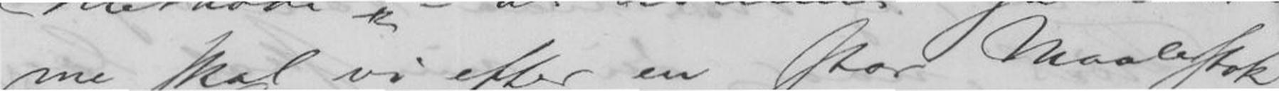me skal vi efter en stor Maalestok
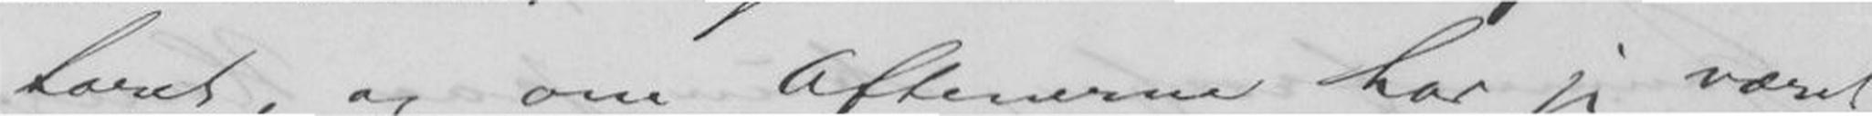toret, og om Aftenerne har jeg været
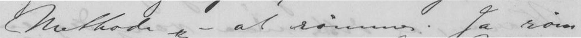Methode - at rømme. Ja røm-
